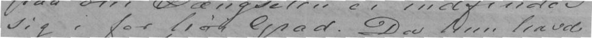sig i for høi Grad. Da hun havde
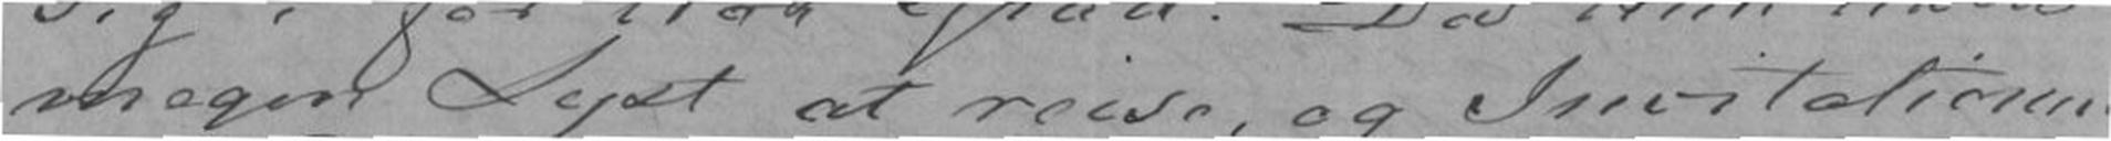megen Lyst at reise, og Invitationen
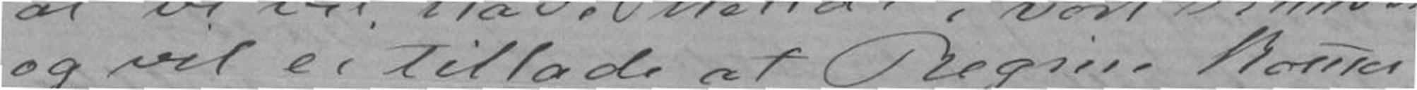og vil ei tillade at Regine ko'mer
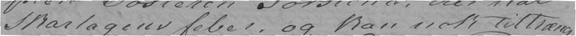Skarlagensfeber, og kan nok tiltrænge
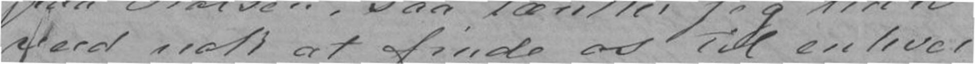veed nok at finde os til enhver
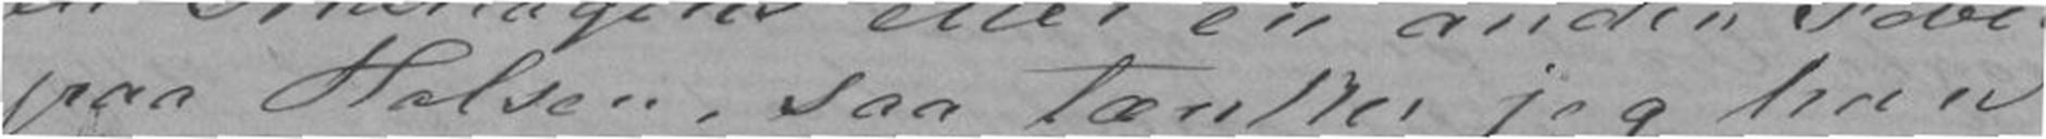paa Halsen, saa tænker jeg han
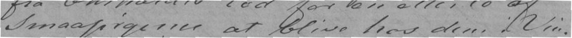Smaapigerne at blive hos dem i Vin-
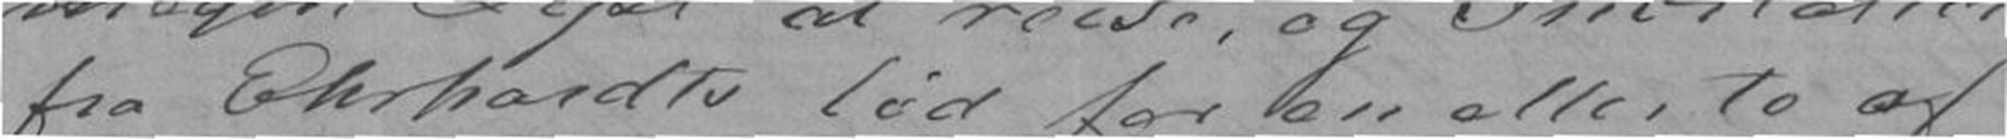fra Ehrhardts lød for en eller to af
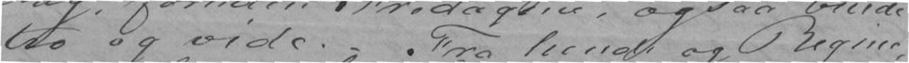tro og vide. - Fra hende og Regine,
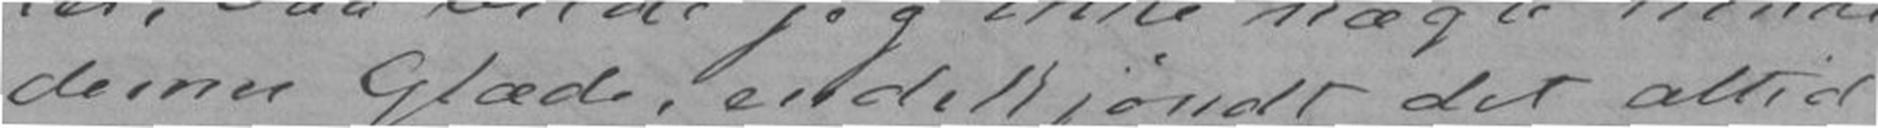denne glæde, endskjøndt det altid
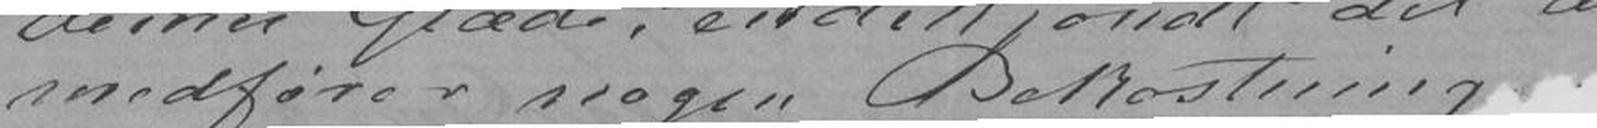medfører nogen Bekostning
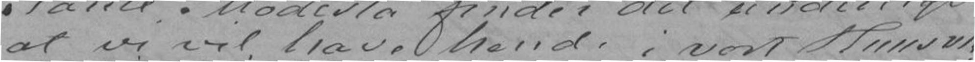at vi vil have hende i vort Huus nu,
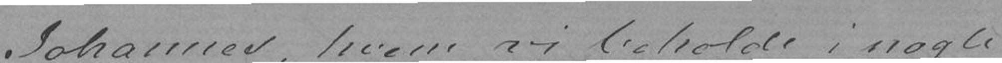Johannes, hvem vi beholde i nogle
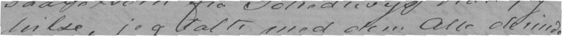hilse, jeg talte med dem Alle derinde
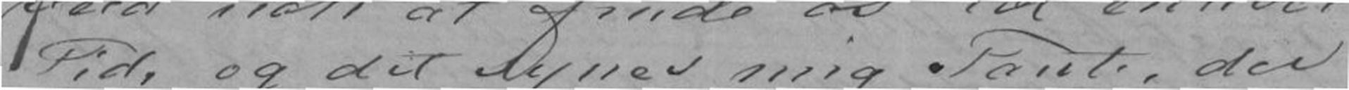Tid, og det synes mig Tante, der
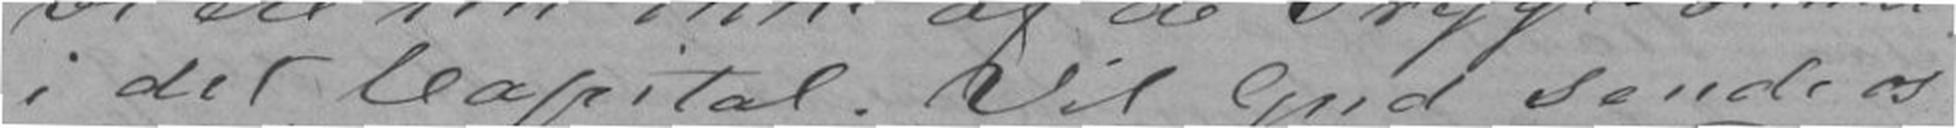i det Capital. Vil Gud sende os
